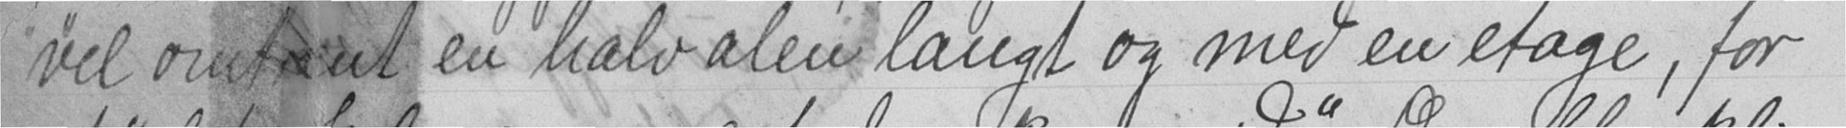vel omtrent en halv alen langt og med en etage, for
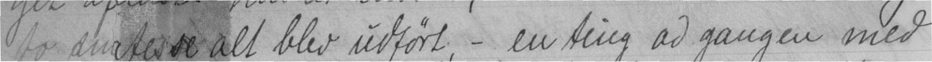to sm atesse alt blev udført, - en ting ad gangen med
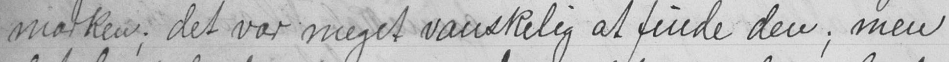marken; det var meget vanskelig at finde den; men
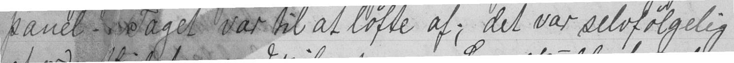panel. Taget var til at løfte af; det var selvfølgelig
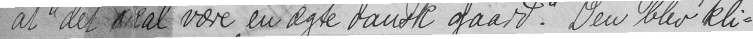at "det skal være en ægte dansk gaard." Den blev kli-
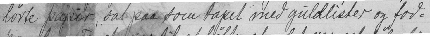lørte papir, sat paa som tapet med guldlister og fod-
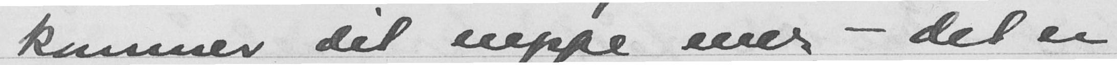kommer dit neppe mer - det er
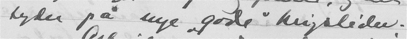tyder på nye "gode" krigstider.
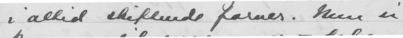i altid skiftende former. Men vi
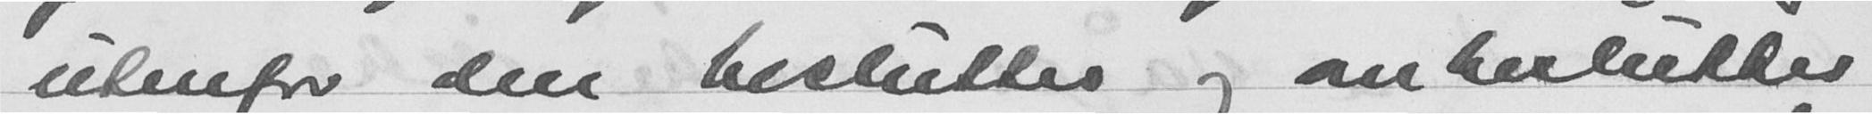utenfor den beslutter og ombeslutter
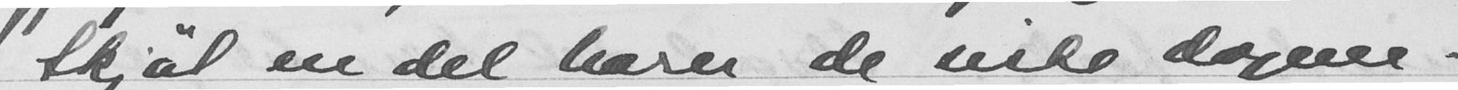Skjøt en del harer de siste dagene.
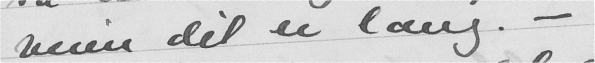veien dit er lang. -
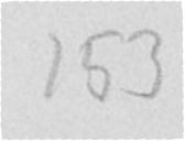153
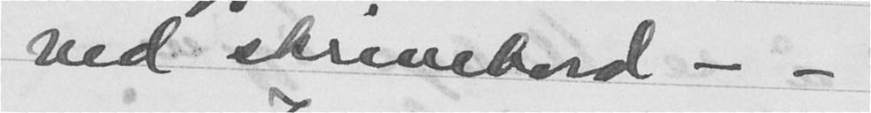ved skrivebord - -
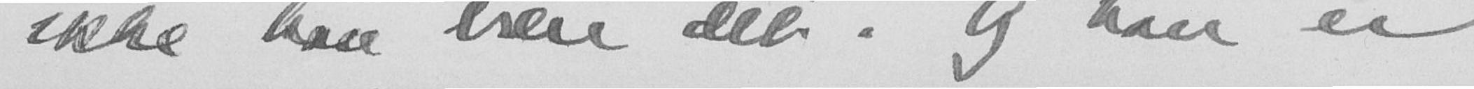ikke han bære det. Og han er
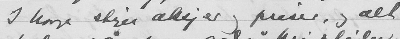I Norge stiger aksjer og priser, og alt
